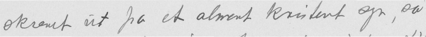skrevet ut fra et alment kristent syn, så
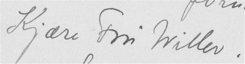Kjære Fru Willer,
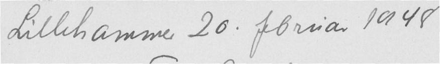Lillehammer 20. februar 1948
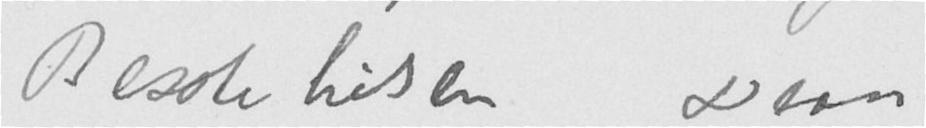Beste hilsen Deres
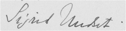Sigrid Undset.
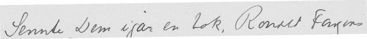Sennte Dem igår en bok, Ronald Fangens
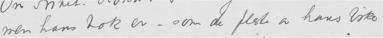men hans bok er - som de fleste av hans bøker
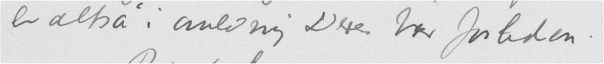er altsa i anledning Deres brev forleden.
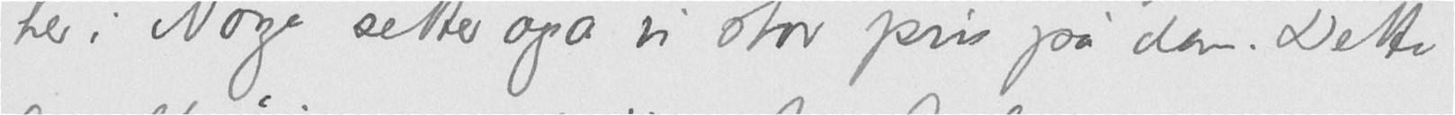her i Norge setter også vi stor pris på den. Dette
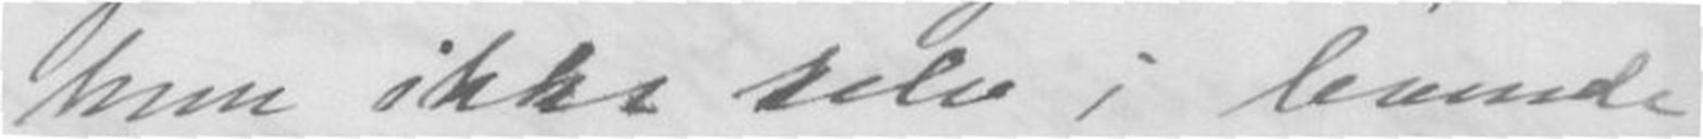hun ikke selv i levende
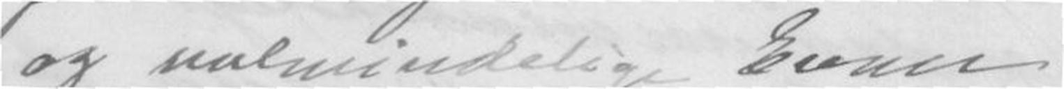og ualmindelig Evner
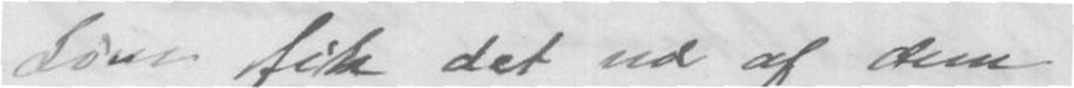Live fik det ud af dem
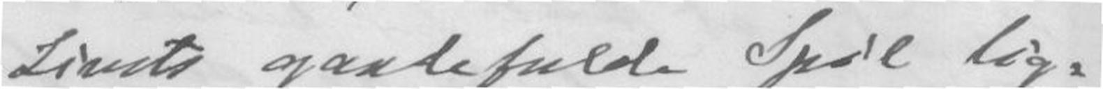livets gaadefulde Spil lig-
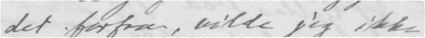det forfra, vilde jeg ikke
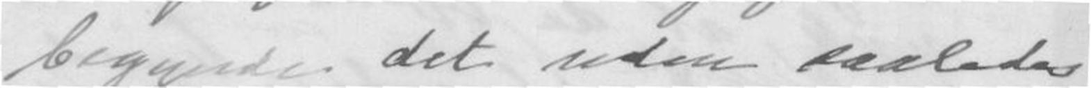begynde det uden saaledes
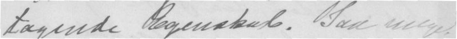tagende Egenskab. Saa meget
